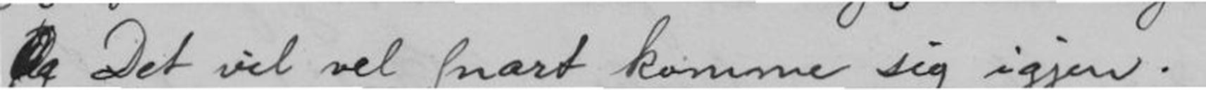Det vil vel snart komme sig igjen.
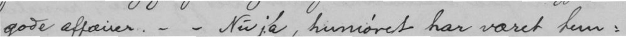gode affærer. - - Nu ja, humøret har været tem-
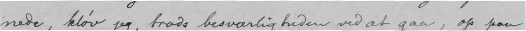nede, kløv jeg, trods besvarligheden ved at gaa, op paa
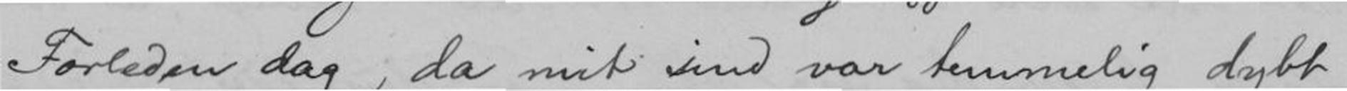Forleden dag, da mit sind var temmelig dybt
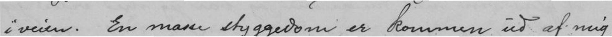i veien. En masse styggedom ud af mig,
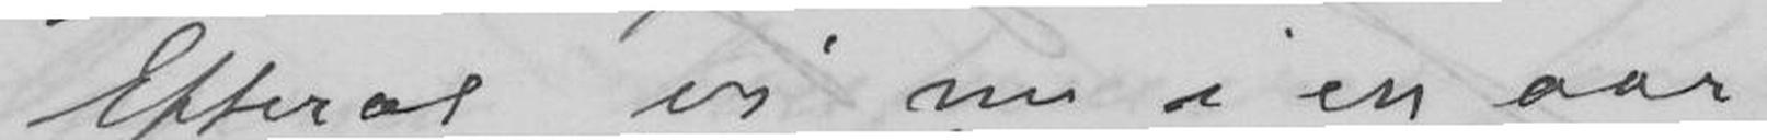Efterat vi nu i en aar-
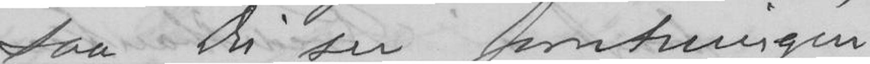saa Du ser, foretningen
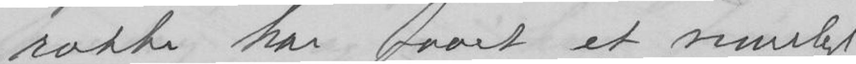række har faaet et rimeligt
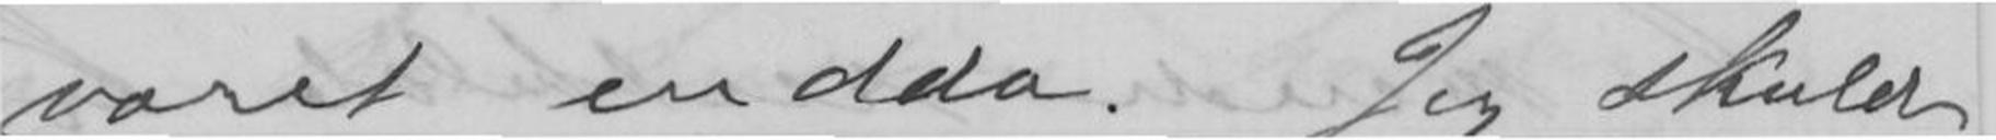værst endda. Jeg skulde
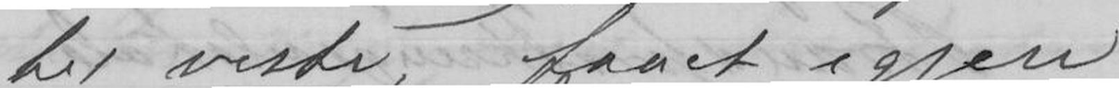bet viste faaet igjen
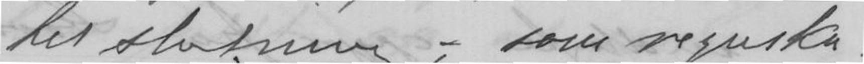til slutning,- som regnska-
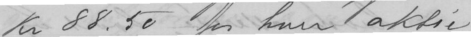kr 88.50 for hver aktie,
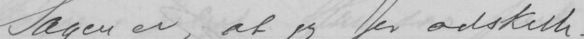Sagen er, at jeg for adskilli-
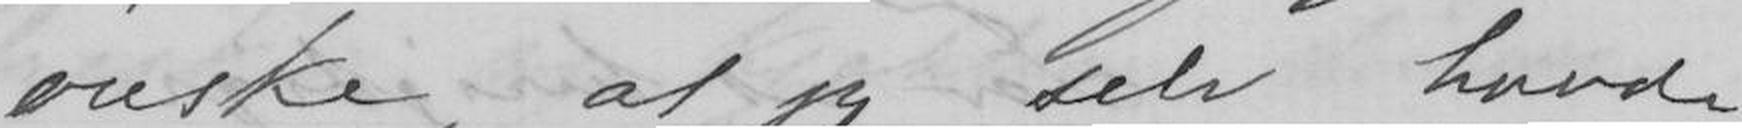ønske, at jeg selv havde
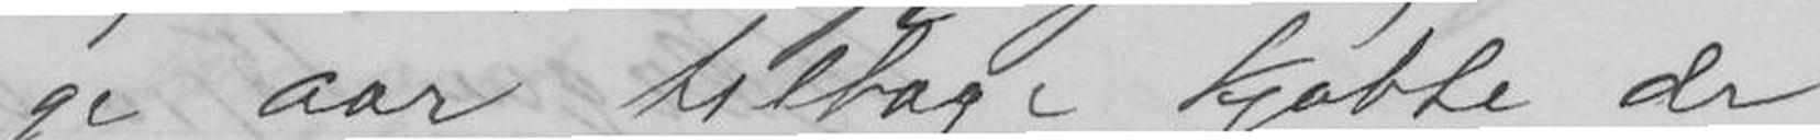ge aar tilbage kjøbte de
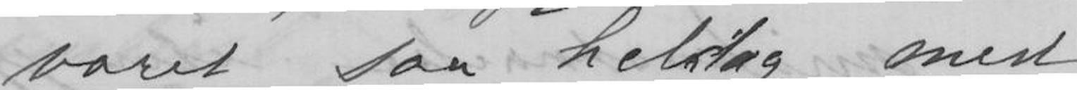været saa heldig med
